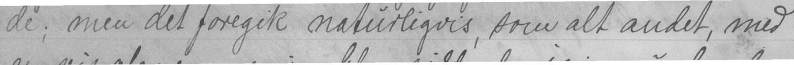de; men det foregik naturligvis, som alt andet, med
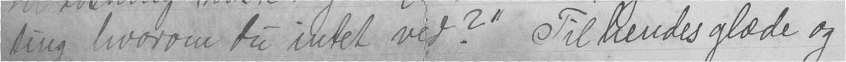ting, hvorom du intet ved?" Til hendes glæde og
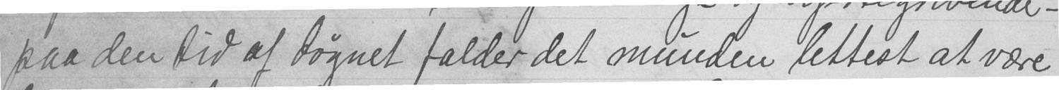paa den tid af døgnet falder det munden lettest at være
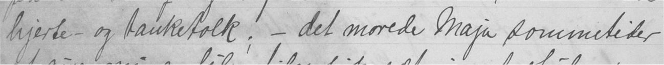hjerte - og tankefolk; - det morede Maja sommetider
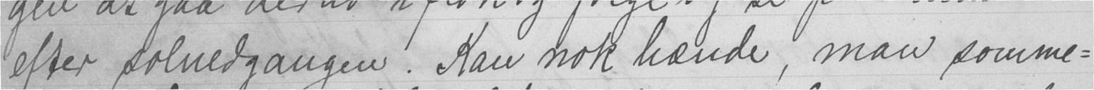efter solnedgangen. Kan nok hænde, man somme-
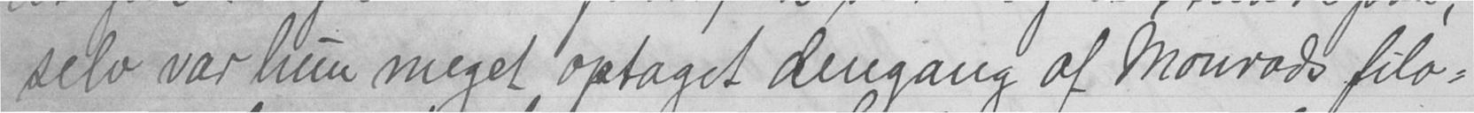selv var hun meget optaget dengang af Monrads filo-
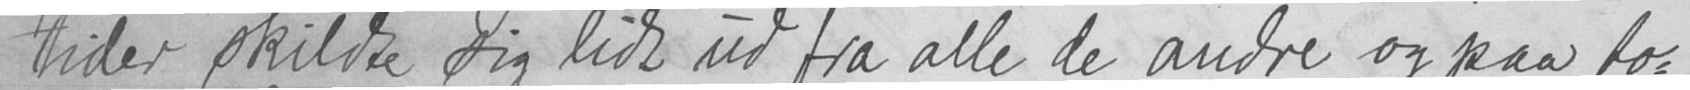tider skildte sig lidt ud fra alle de andre og paa to-
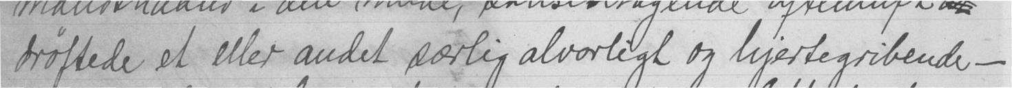drøftede et eller andet særlig alvorligt og hjertegribende -
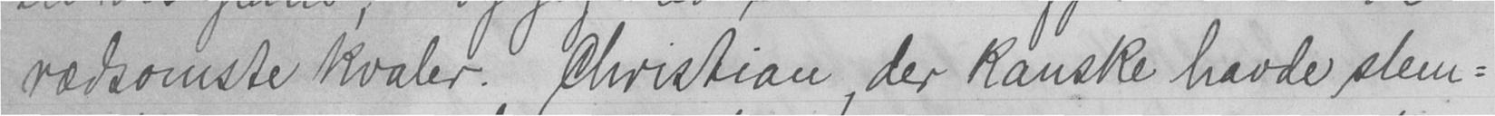rædsomste kvaler. Christian, der kanske havde slem-
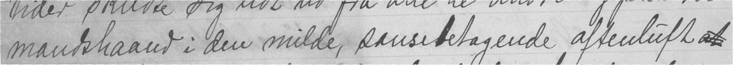mandshaand i den milde, sansebetagende aftenluft at
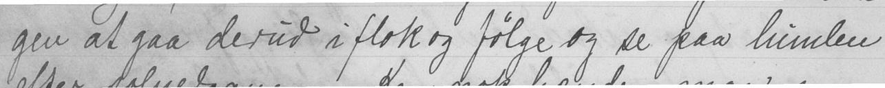gen at gaa derud i flok og følge og se paa himlen
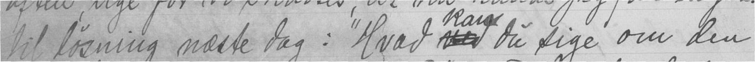til løsning næste dag: "Hvad ved  kan du sige om den
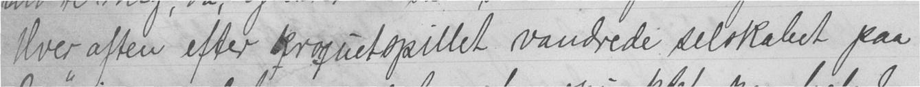Hver aften efter kroquetspillet vandrede selskabet paa
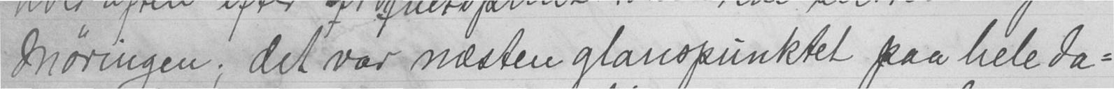Møringen; det var næsten glanspunktet paa hele da-
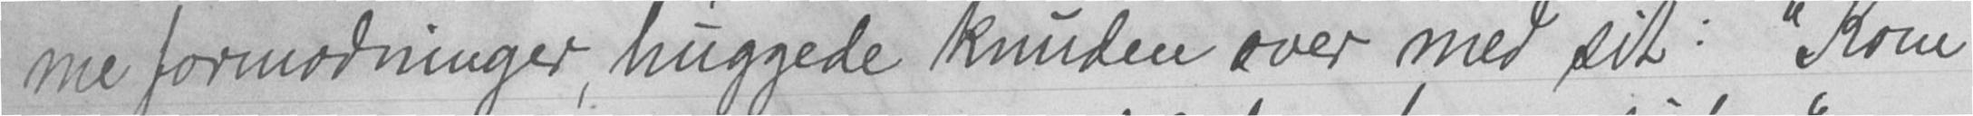me formodninger, huggede knuden over med sit: "Kom
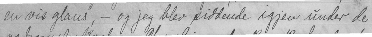en vis glans, - og jeg blev siddende igjen under de
